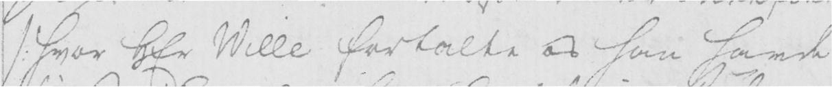/: Hvor Hr Wille fortalte os han havde
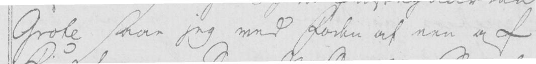Grote saae jeg ved Foden af een af
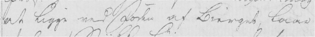at ligge ved Foden at Bierget laae
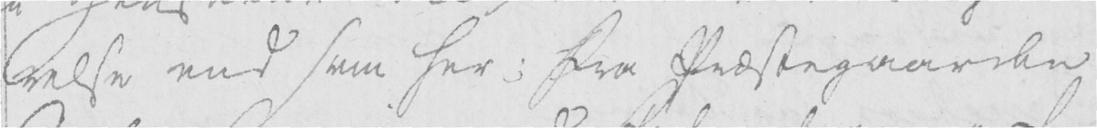relse end som her; fra Præstegaarden
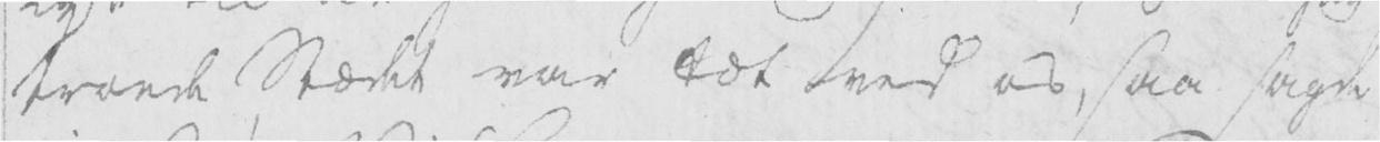troede Stædet var tæt ved os, saa sagde
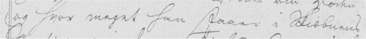og hvor meget han staaer i Skiæbnens
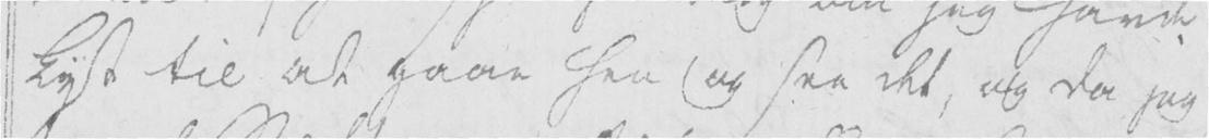Lyst til at gaae hen og see det, og da jeg
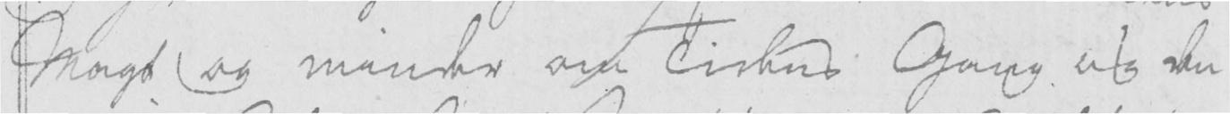Magt og minder om Tidens Gang og den
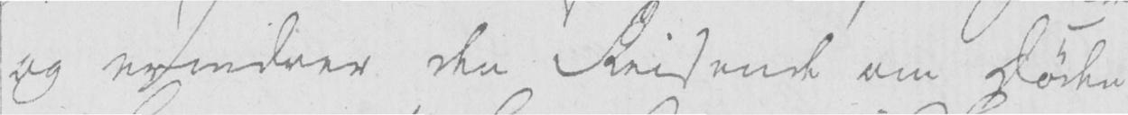og erindrer den Reisende om Døden
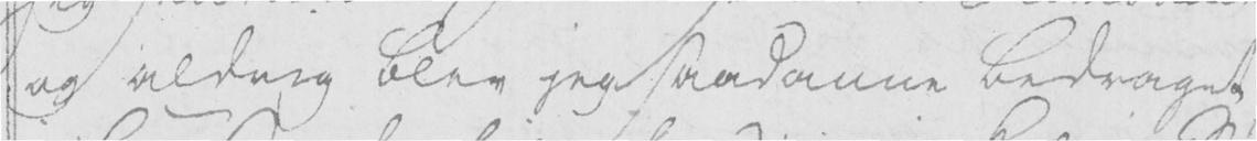og aldrig blev jeg saadanne bedraget
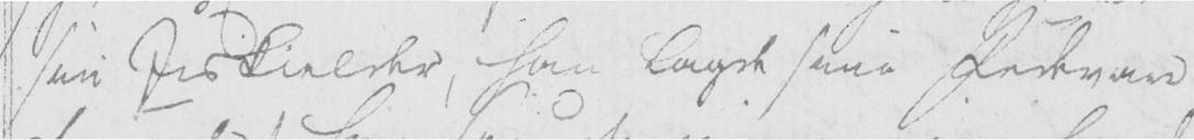sin Iiskielder, han lagde sine Fedevare
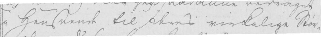i Henseende til deres virkelige Stør-
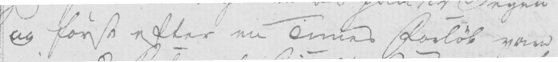og først efter en Times Forløb vare
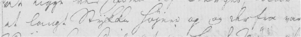at langt Stykke højere op og derfra var
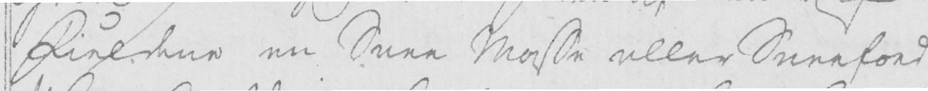Fieldene en Snee Masse eller Sneefond
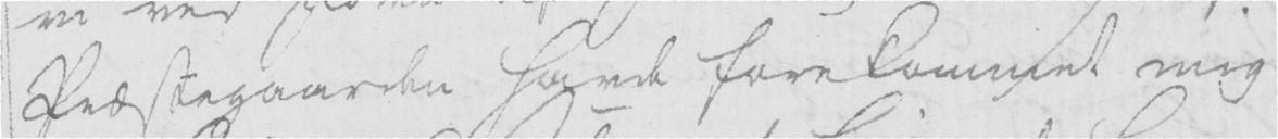Præstegaarden havde forekommet mig
